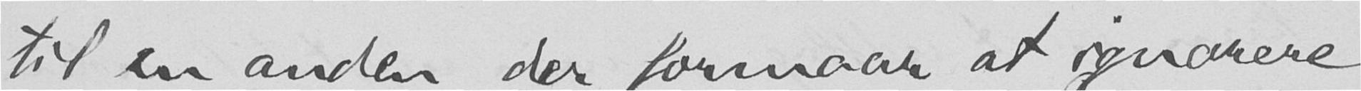til en anden, der formaar at ignorere
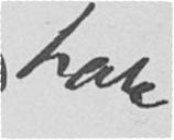har
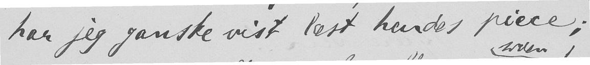har jeg ganske vist læst hendes piece;
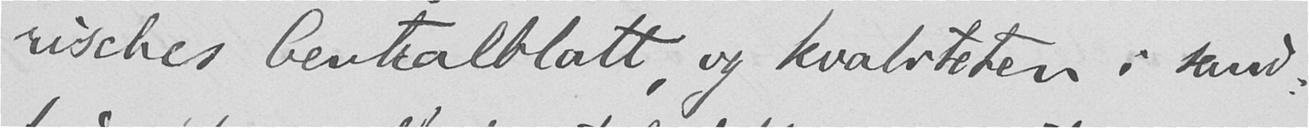risches Centralblatt, og kvaliteten i sand-
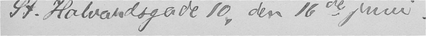St. Halvardsgade 10, den 16de juni.
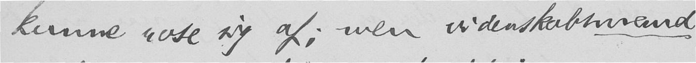kunne rose sig af; men videnskabsmand
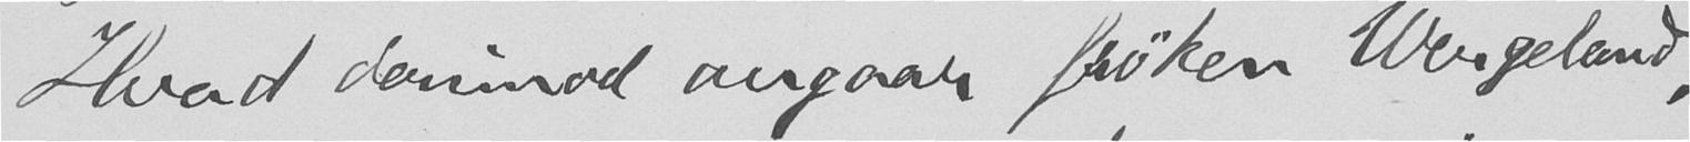Hvad derimod angaar frøken Wergeland,
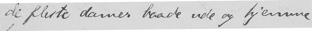de fleste damer baade ude og hjemme
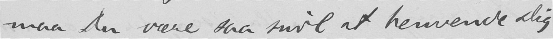maa Du være saa snil at henvende Dig
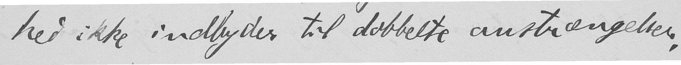hed ikke indbyder til dobbelte anstrængelser,
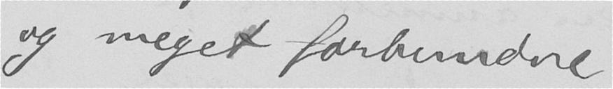og meget forbundne
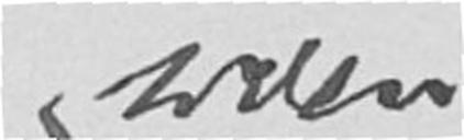siden
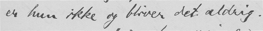er hun ikke og bliver det aldrig.
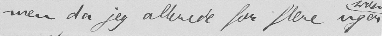men da jeg allerede for flere uger
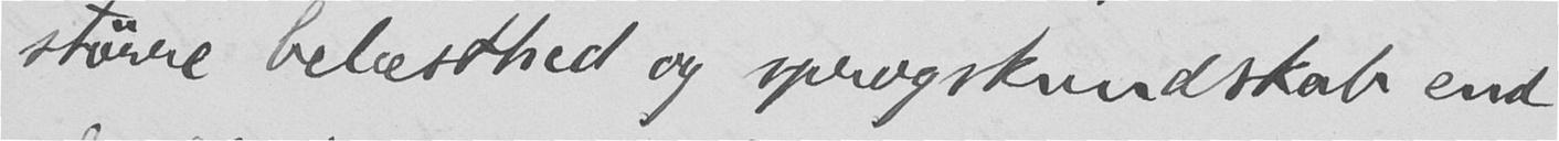større belæsthed og sprogskundskab end
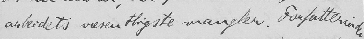arbeidets væsentligste mangler. Forfatterinden
